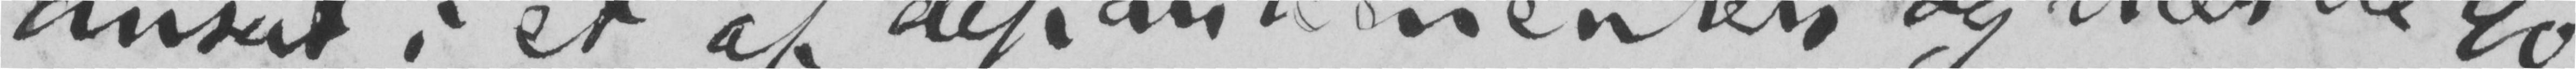ansat i et af deptartementene og nær de 40.
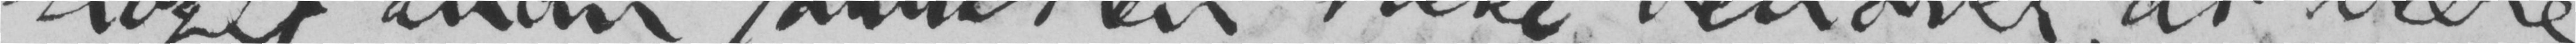noget man forrester ikke behøver at være
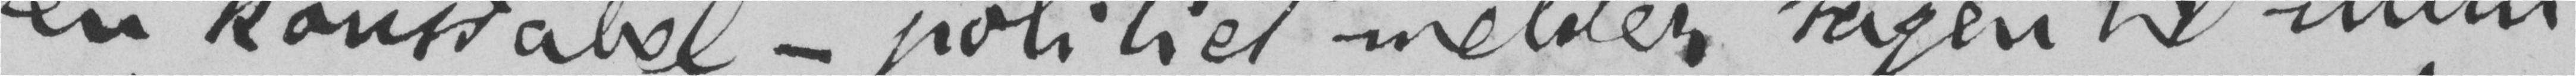en konstabel – politiet melder sagen til mini-
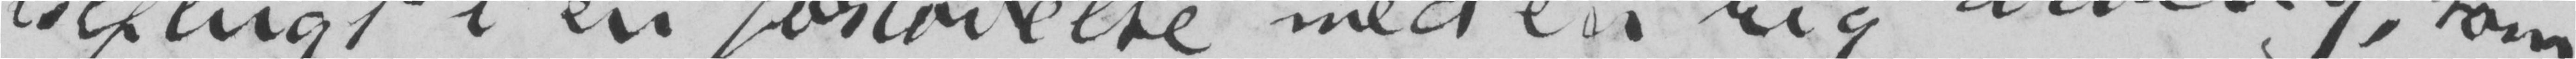tilflugt i en forlovelse med en rig arving, som
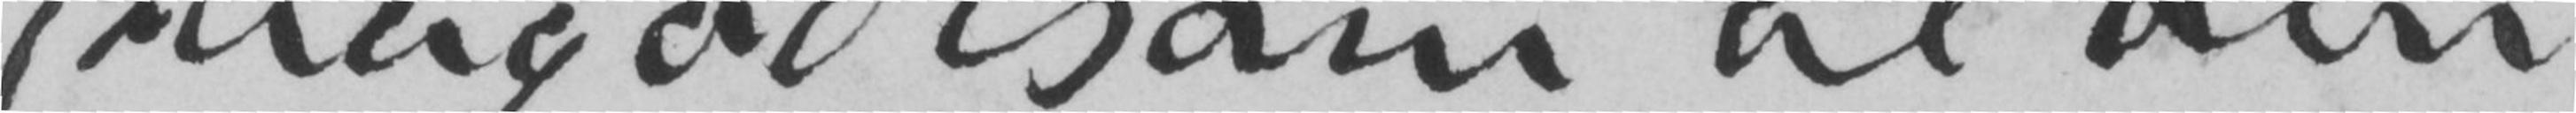saagodtsom al om-
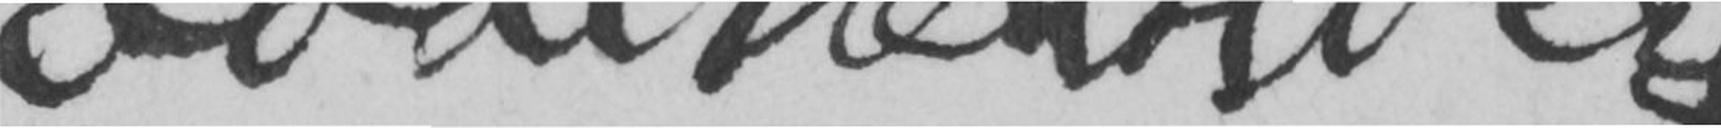Ebbe Hertzberg.
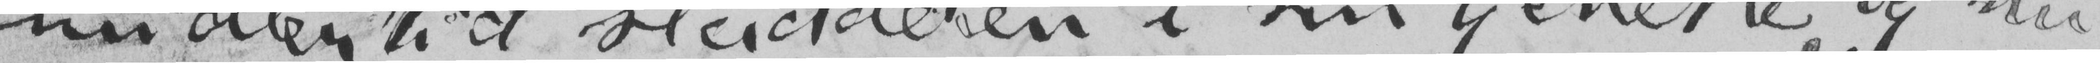midlertid sladderen i sin tjeneste, og nu
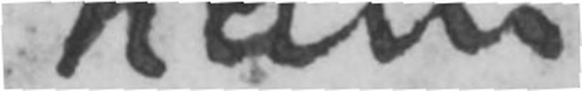ham
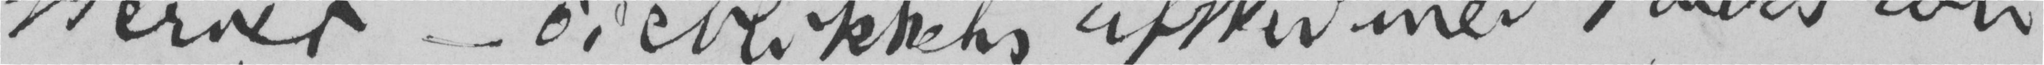steriet – øieblikkelig afsked med 1 aars løn
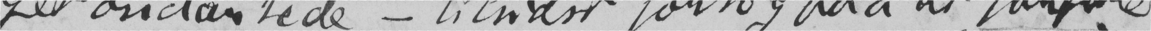get ondartede – tilsidst forsøg paa at forføre
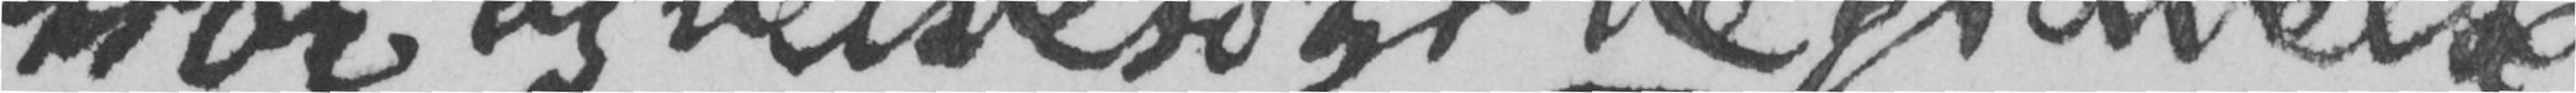stor og velbesøgt begravelse.
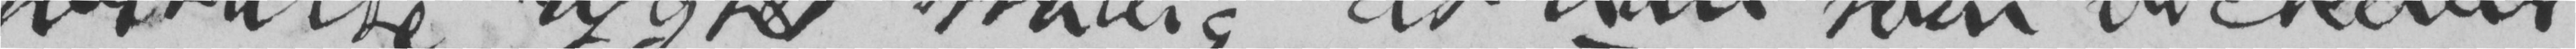fortalte rygtet stadig, at han som vilkaar
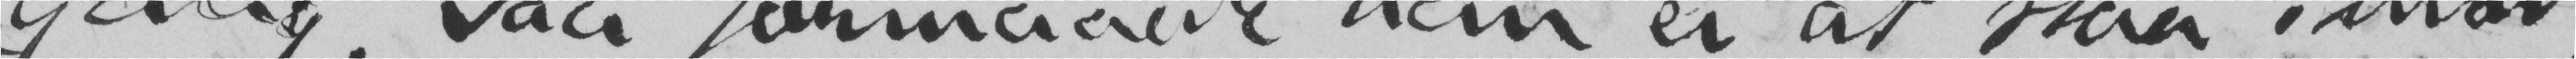gang. Saa formaaede han ei at staa imod
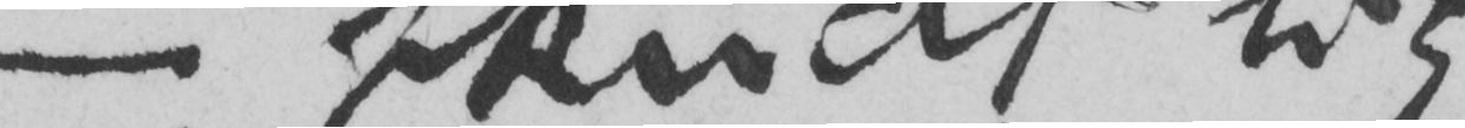–  skudt sig –
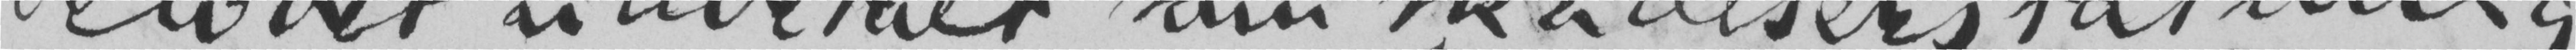beløbet udbetalt som skadeserstatning,
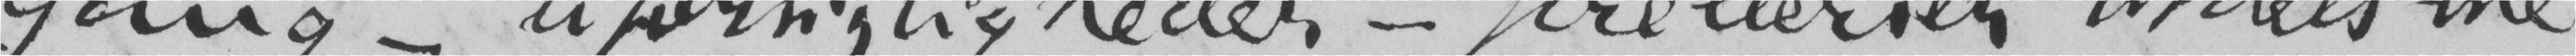gang – uforsigtigheder – prellerier, til dels me-
